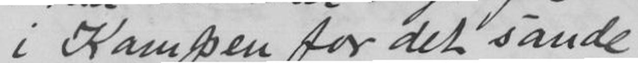i Kampen for det sande,
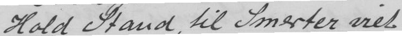Hold Stand, til Smerter viet
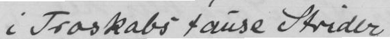i Troskab tause Strider,
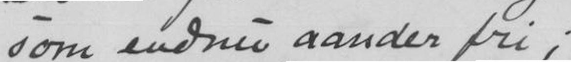som endnu aander fri;
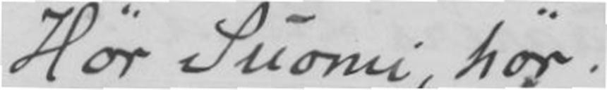Hør Suomi, hør!
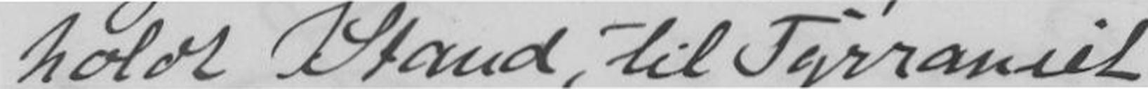holdt Stand, til Tyrraniet
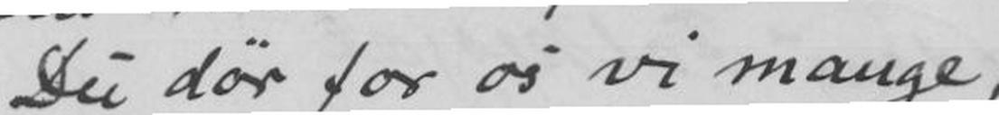Du dør for os vi mange,
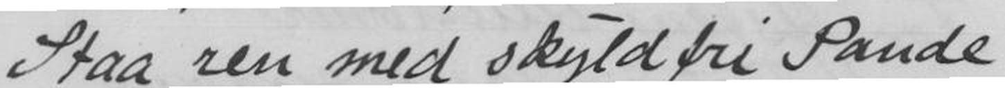Staa ren med skyldfri Sande
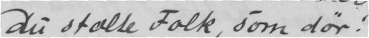Du stolte Folk, som dør!
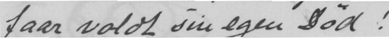faar voldt sin egen Død!
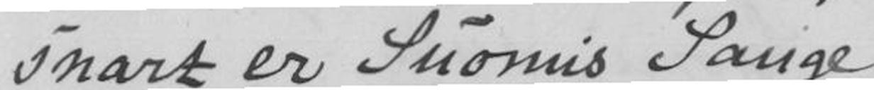snart er Suomis Sange
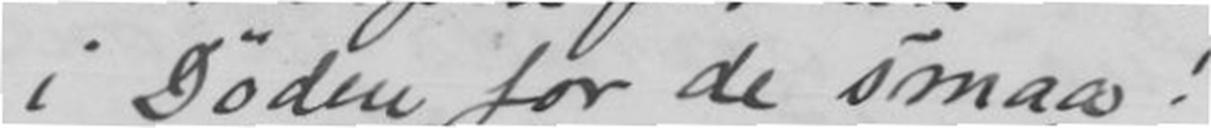i Døden for de smaa!
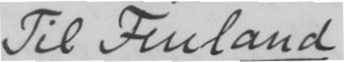Til Finland
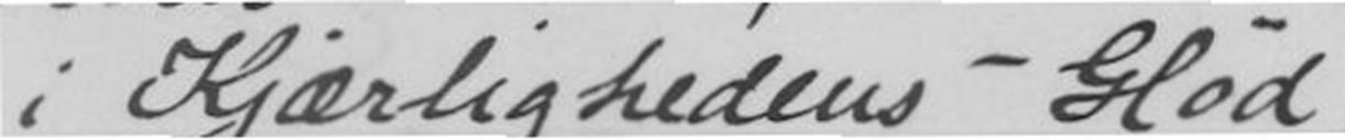i Kjærlighedens - Glød,
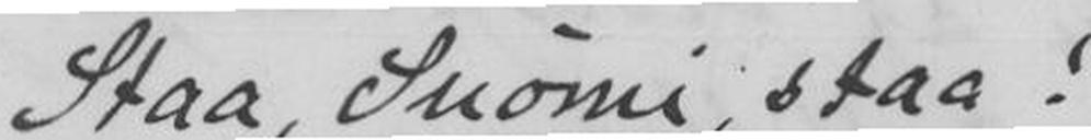Staa, Suomi, staa!
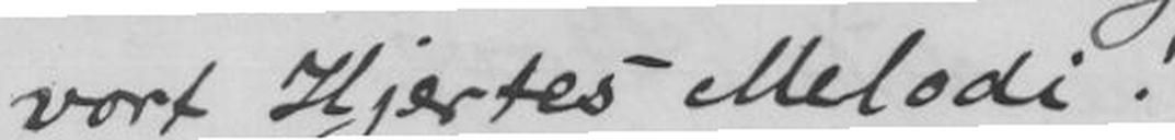vort Hjertes Melodi!
